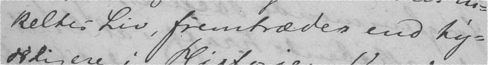keltes Liv, fremtræder end ty-
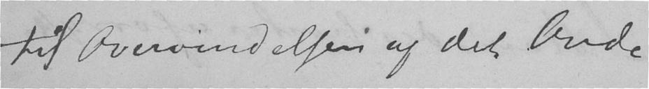til Overvindelsen af det Onde
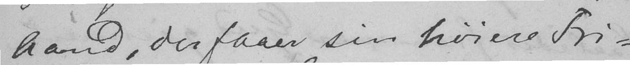Aand, der faaer sin høiere Fri-
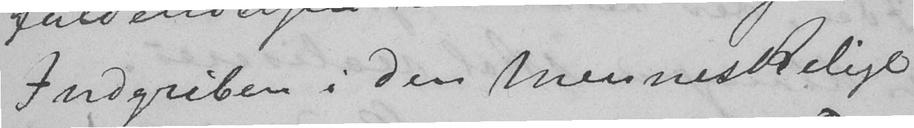Indgriben i den menneskelige
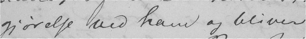gjørelse ved ham og bliver
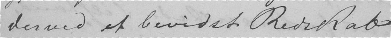derved et bevidst Redskab
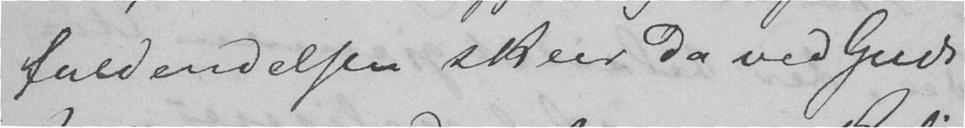fuldendelsen skeer da ved Guds
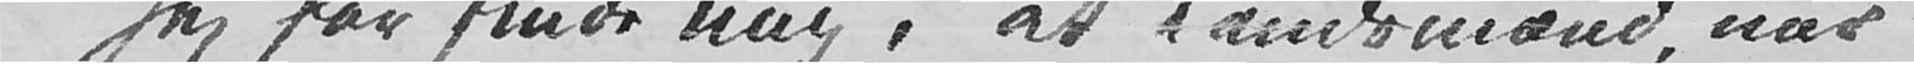Jeg får finde mig i at Landsmænd, når
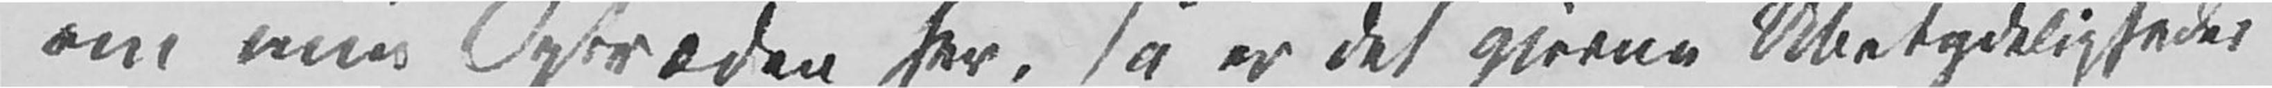om min Optræden her, så er det gjerne Ubetydeligheder
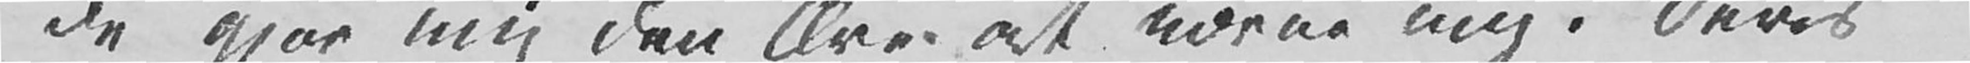de gjør mig den Ære at nævne mig i Deres
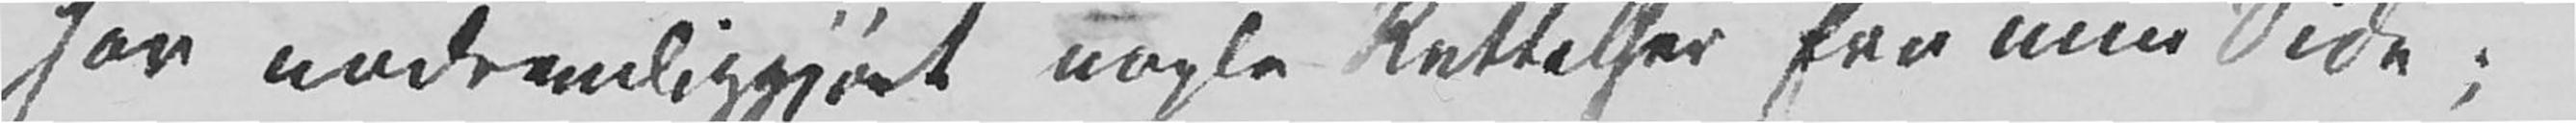har nødvendiggjort nogle Rettelser fra min Side;
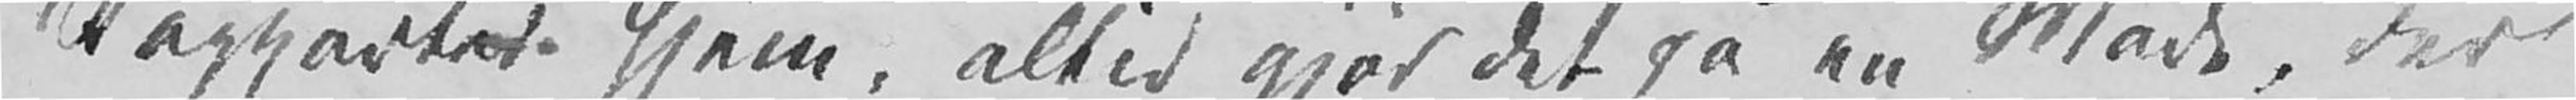Rapporter hjem, altid gjør det på en Måde, der
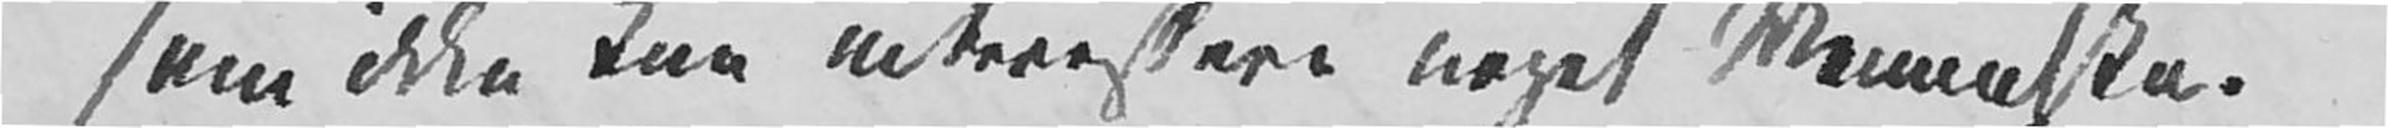som ikke kan interessere noget Menneske.
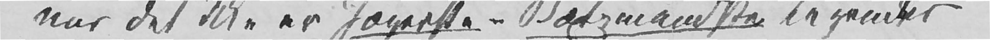når det ikke er Jægerske-Bætzmandske Legender
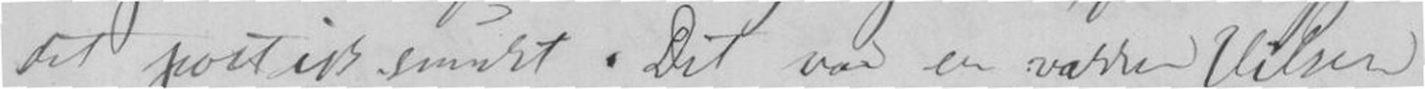det poetisk smukt. Det var en vakker Hilsen
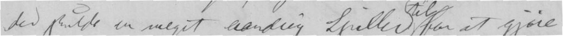der skulde en meget aandrig Spiller for at gjøre
